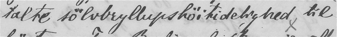talte sølvbryllupshøitidelighed til
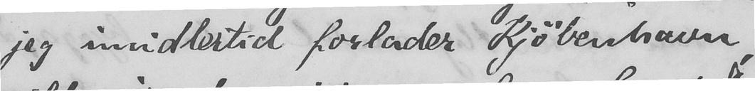jeg imidlertid forlader Kjøbenhavn,
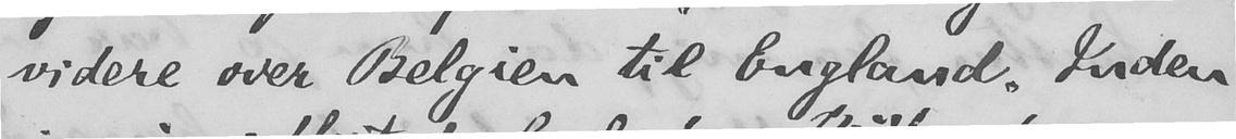videre over Belgien til England. Inden
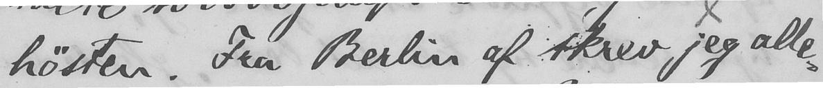høsten. Fra Berlin af skrev jeg alle-
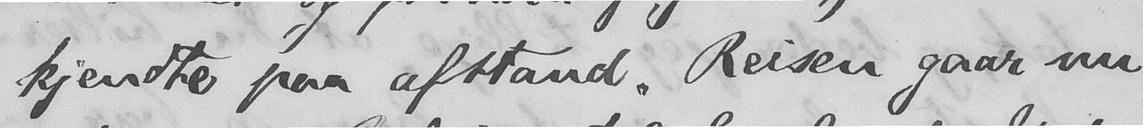kjendte paa afstand. Reisen gaar nu
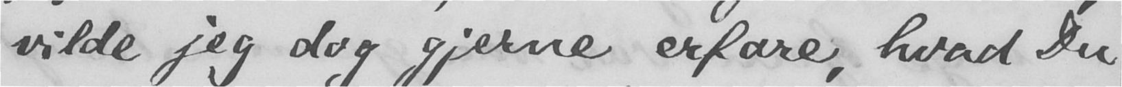vilde jeg dog gjerne erfare, hvad Du
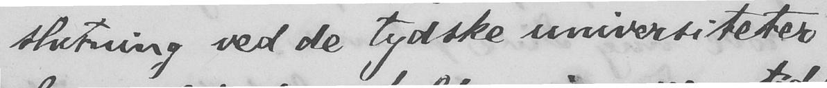slutning ved de tydske universiteter
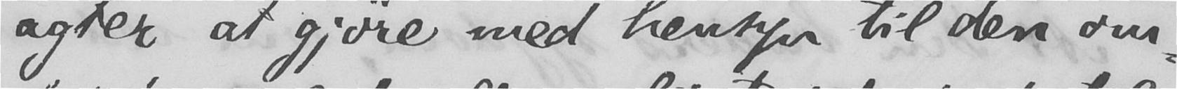agter at gjøre med hensyn til den om-
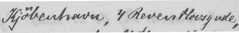Kjøbenhavn, 4 Reventlovsgade
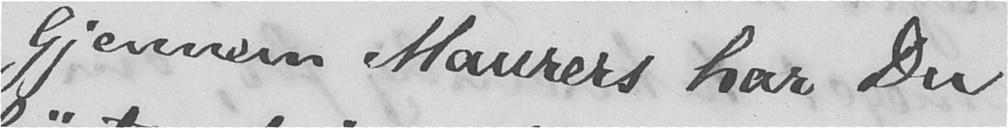Gjennem Maurers har Du
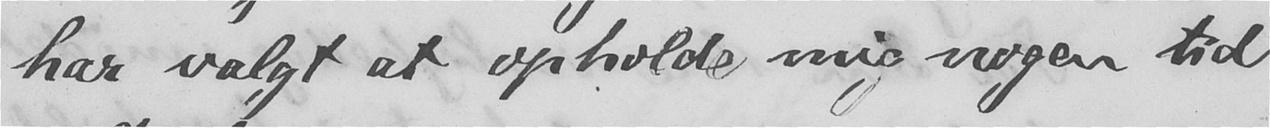har valgt at opholde mig nogen tid
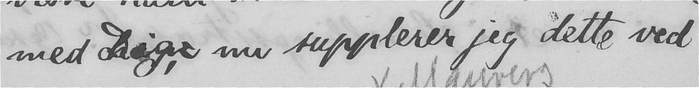med Dig; nu supplerer jeg dette ved
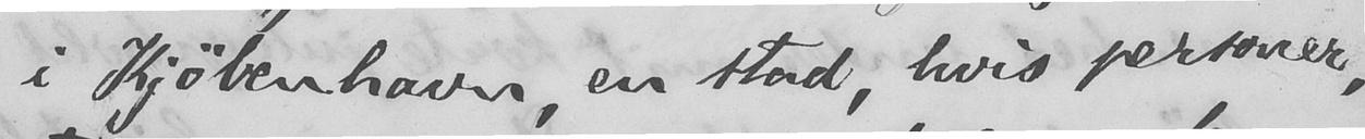i Kjøbenhavn, en stad, hvis personer,
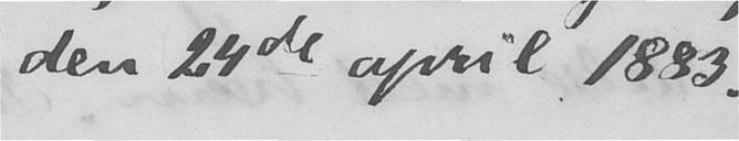den 24de april 1883.
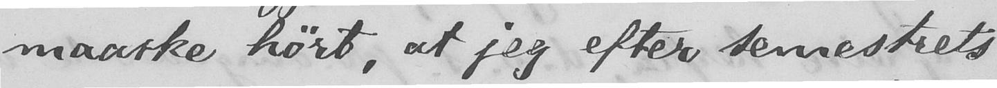maaske hørt, at jeg efter semestrets
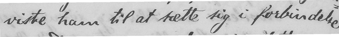viste ham til at sætte sig i forbindelse
